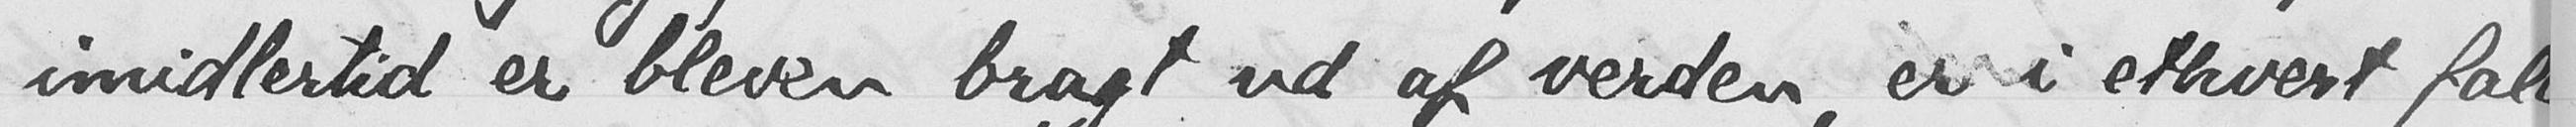imidlertid er bleven bragt ud af verden, er i ethvert fald
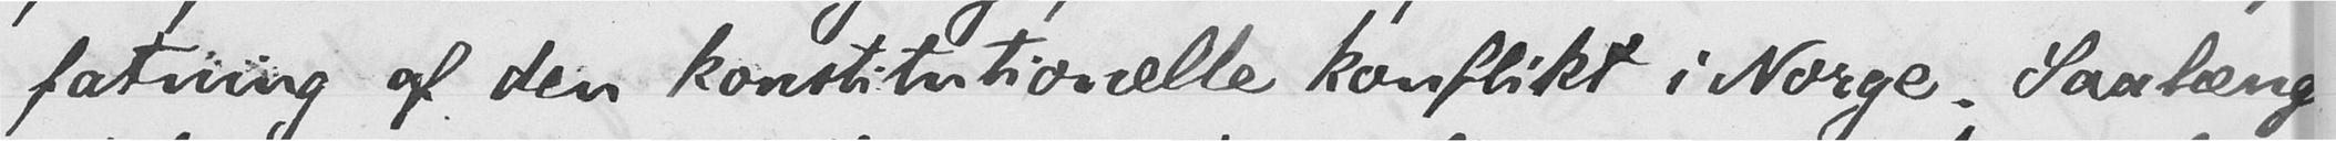fatning af den konstitutionelle konflikt i Norge. Saalænge
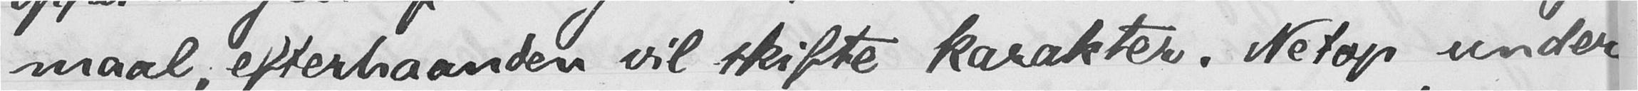maal, efterhaanden vil skifte karakter. Netop under
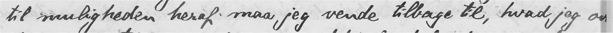til muligheden heraf maa jeg vende tilbage til, hvad jeg oven-
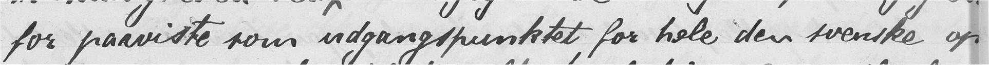for paaviste som udgangspunktet for hele den svenske op-
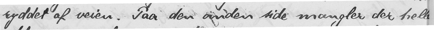ryddet af veien. Paa den anden side mangler der heller
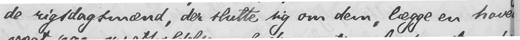de rigsdagsmænd, der slutte sig om dem, lægge en hoved-
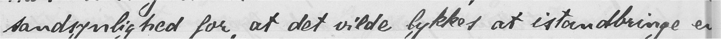sandsynlighed for, at det vilde lykkes at istandbringe en
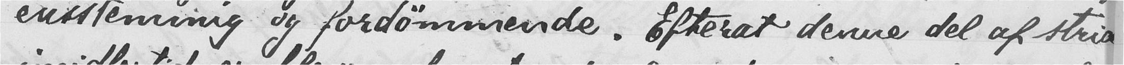ensstemmig og fordømmende. Efterat denne del af striden
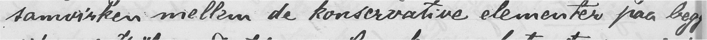samvirken mellem de konservative elementer paa begge
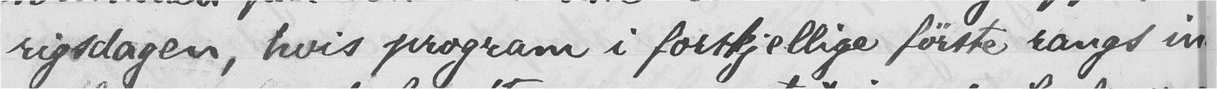rigsdagen, hvis program i forskjellige første rangs indre
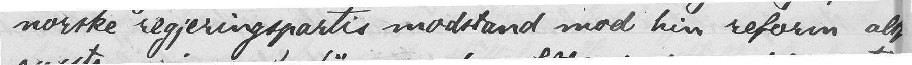norske regjeringspartis modstand mod hin reform altfor
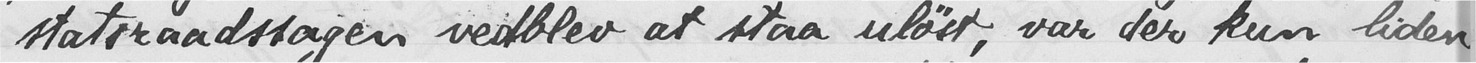statsraadssagen vedblev at staa uløst, var der kun liden
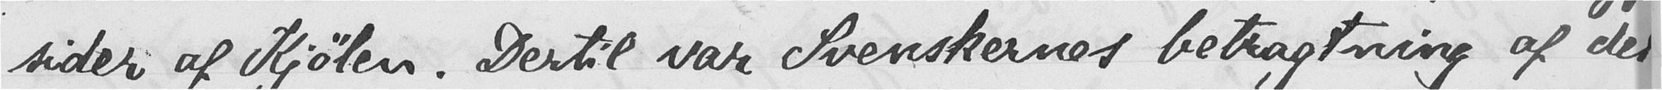sider af Kjølen. Dertil var Svenskernes betragtning af det
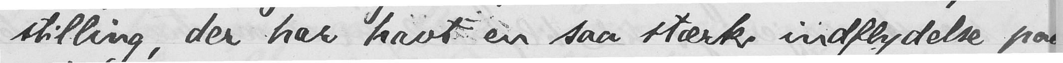stilling, der har havt en saa stærk indflydelse paa
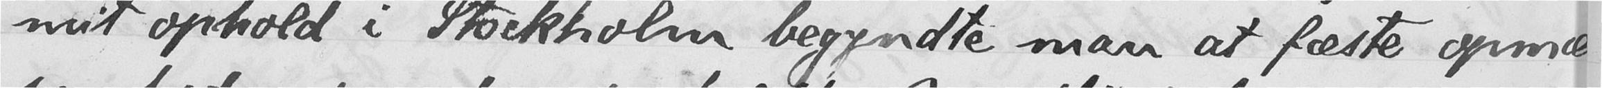mit ophold i Stockholm begyndte man at fæste opmærk-
